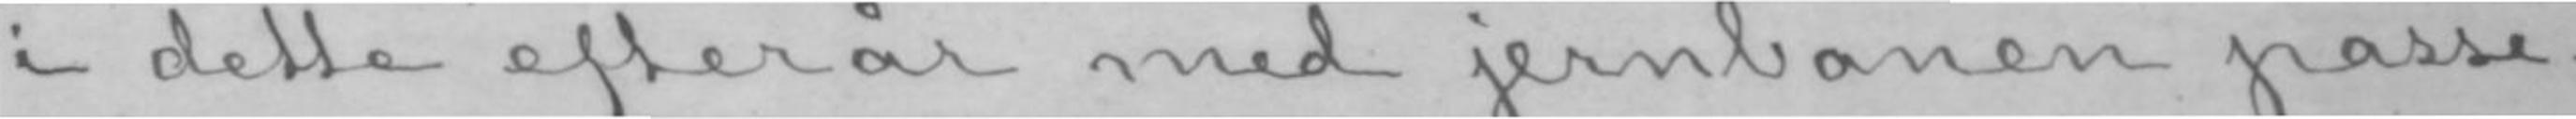i dette efterår med jernbanen passe-
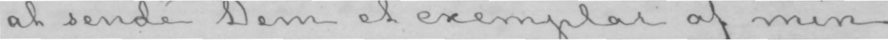at sende Dem et exemplar af min
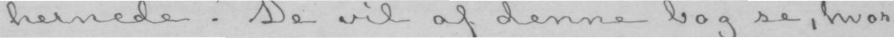hernede. De vil af denne bog se, hvor-
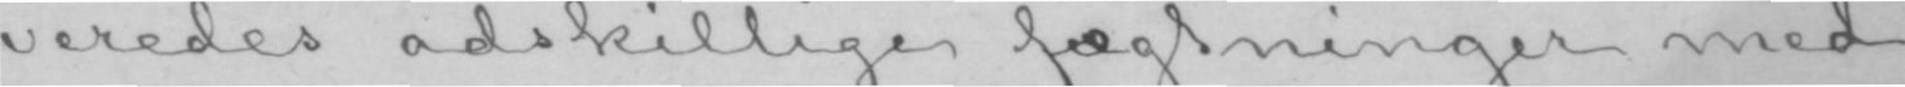veredes adskillige fægtninger med
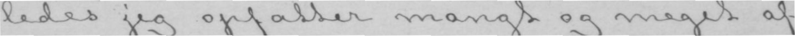ledes jeg opfatter mangt og meget af
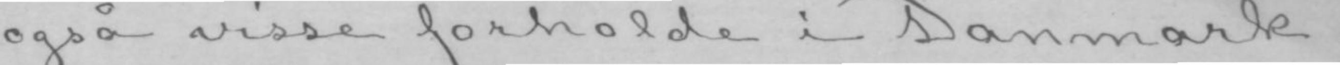også visse forholde i Danmark.
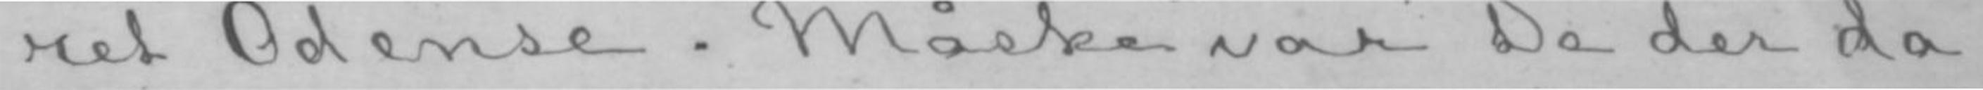ret Odense. Måske var De der da,
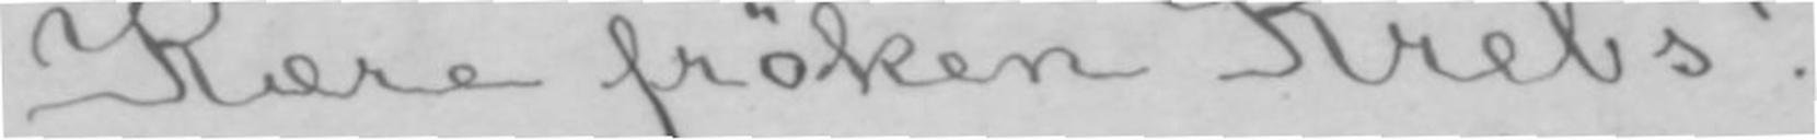Kære frøken Krebs!
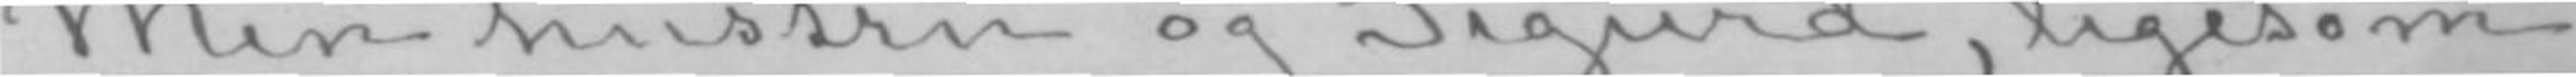Min hustru og Sigurd, ligesom
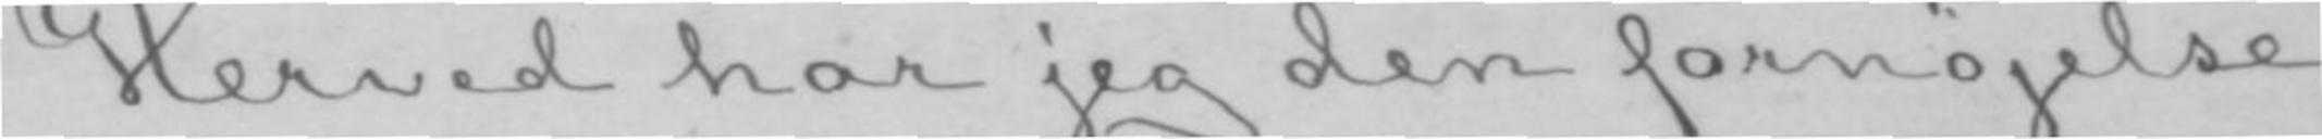Herved har jeg den fornøjelse
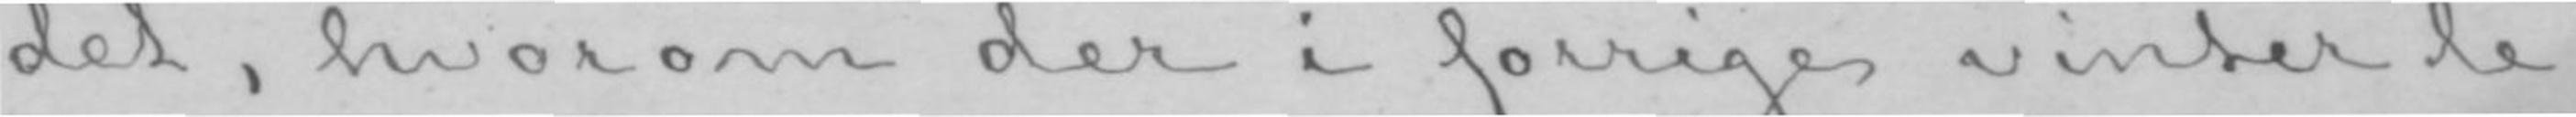det, hvorom der i forrige vinter le-
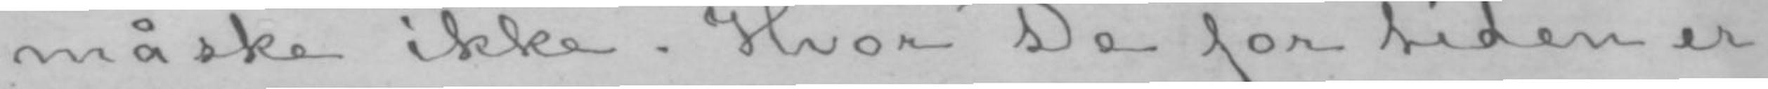måske ikke. Hvor De for tiden er,
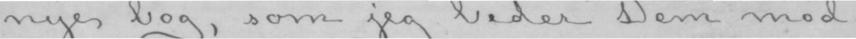nye bog, som jeg beder Dem mod-
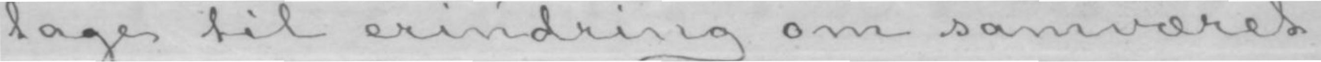tage til erindring om samværet
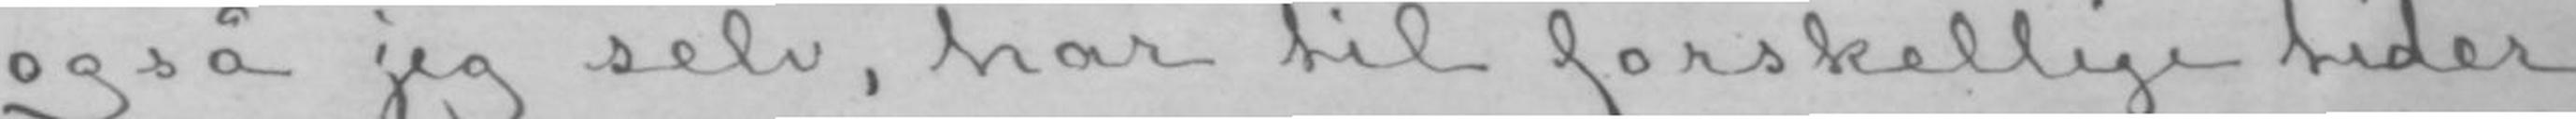også jeg selv, har til forskellige tider
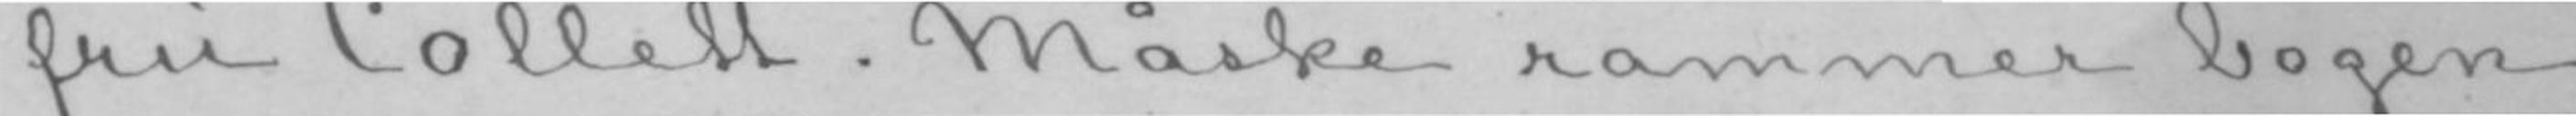fru Collett. Måske rammer bogen
